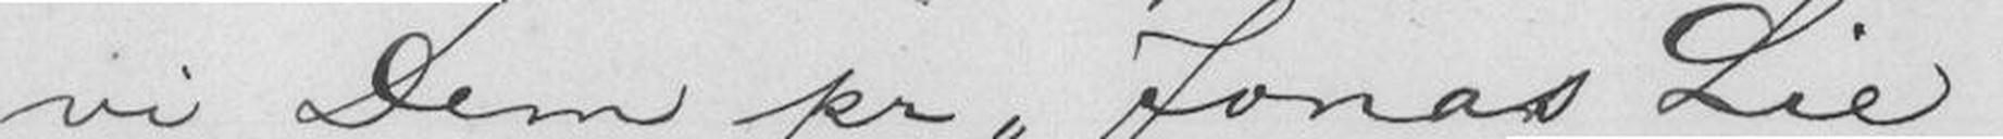vi Dem pr "Jonas Lie"
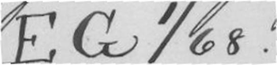EG 1/68.
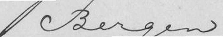Bergen
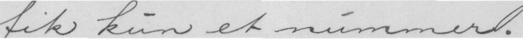fik kun et nummer.
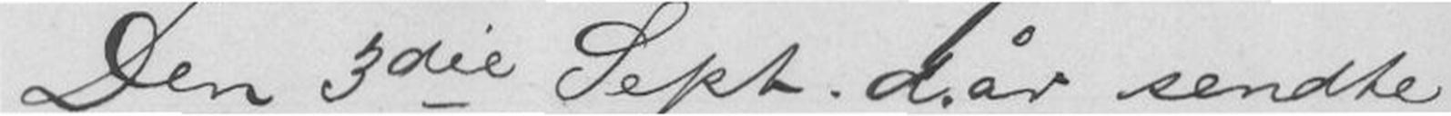Den 3die Sept. d. år sendte
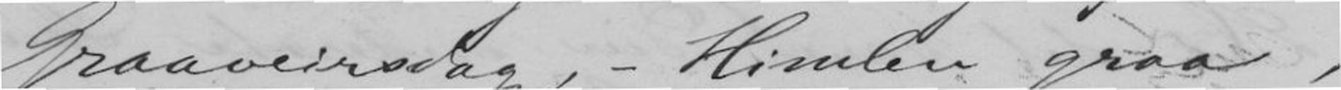Graaveirsdag, - Himlen graa,
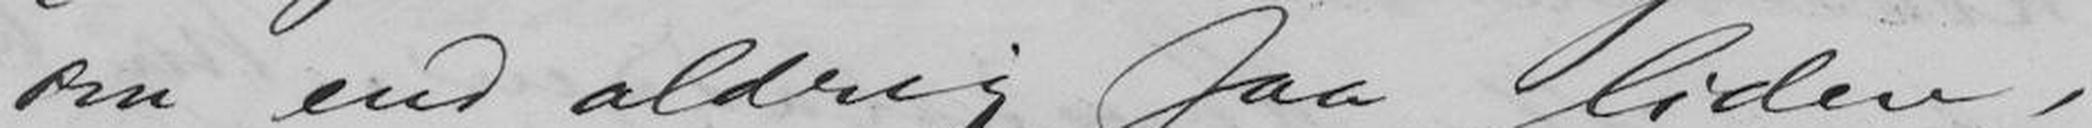om end aldrig saa liden,
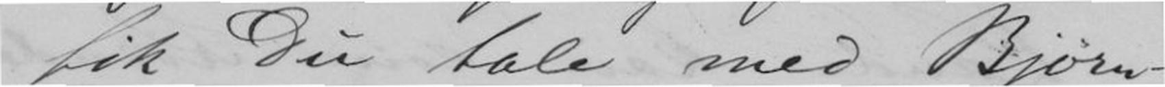fik Du tale med Bjørn-
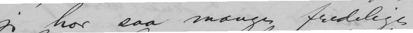jeg har saa mange fredelige
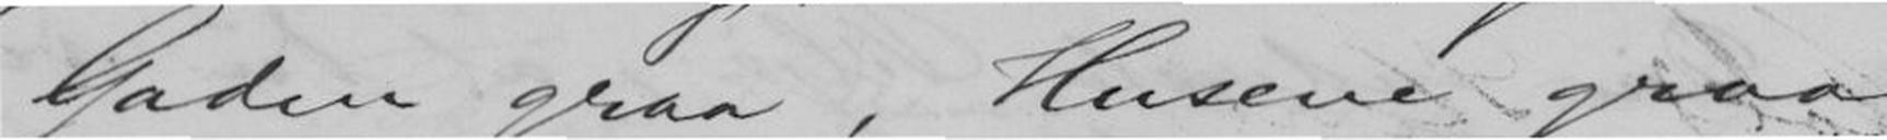Gaden graa, Husene graa
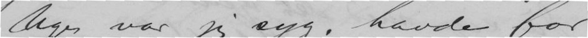Uge var jeg syg, havde for-
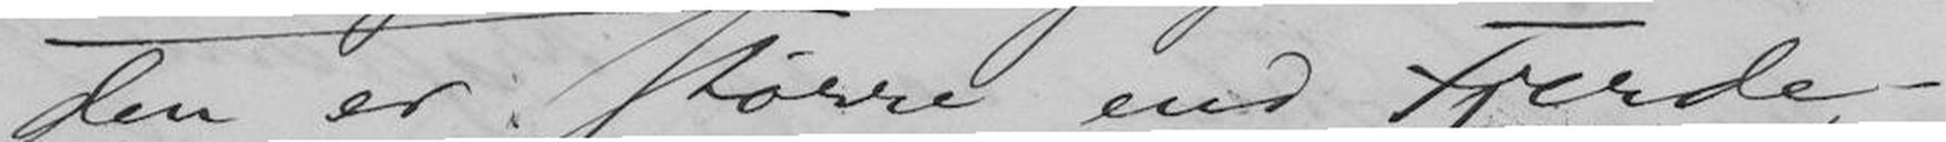den er større end Fjerde-
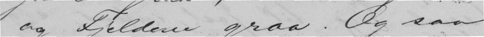og Fjeldene graa. Og saa
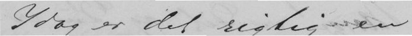Idag er det rigtig en
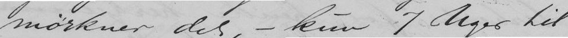mørkner det, - kun 7 Uger til
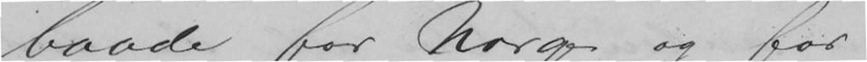baade for Norge og for
